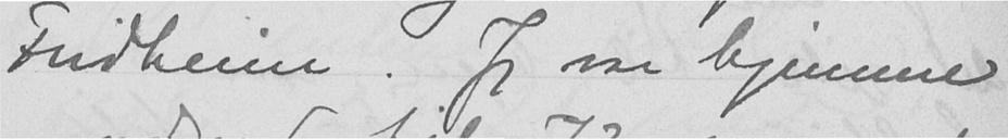Fridheim. Jeg var hjemme
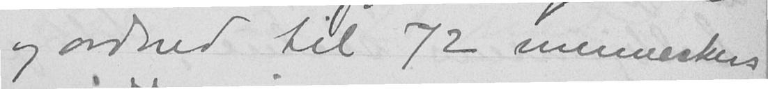og ordned til 72 menneskers
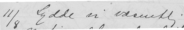11/8 Sydde vi væsentlig
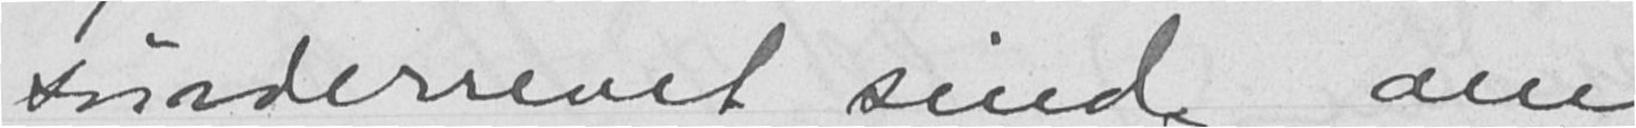sønderrevet sind om
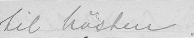til høsten.
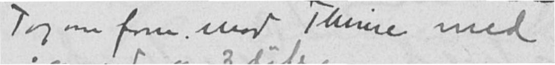Tog om form. mod Thrine med
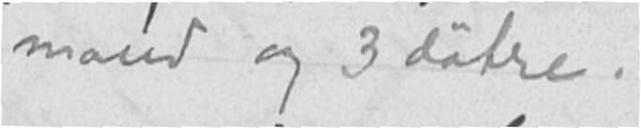mand og 3 døtre.
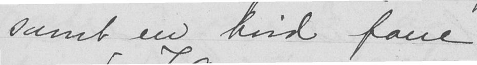samt en hvid fane
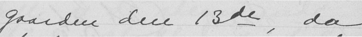gaarden den 13de, da
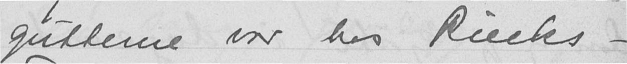gutterne var hos Riecks -
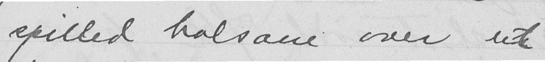spilled balsam over ut
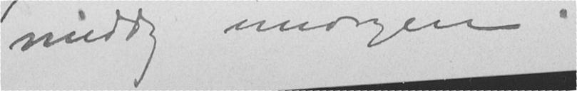middag imorgen.
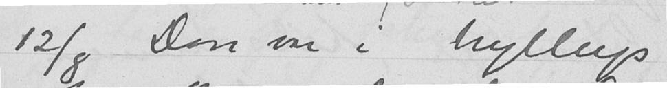12/8 Don var i bryllup
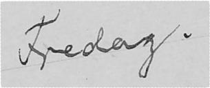Fredag.
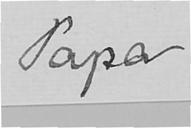Papa
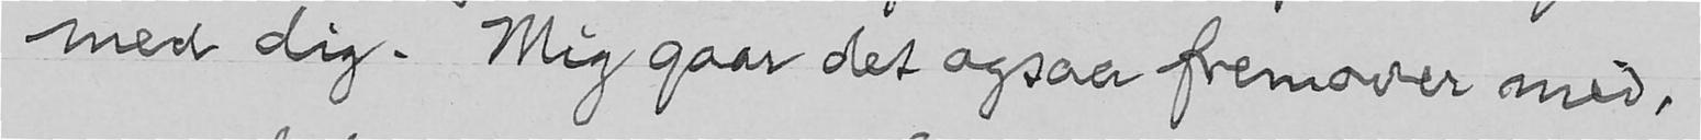med dig. Mig gaar det ogsaa fremover med,
